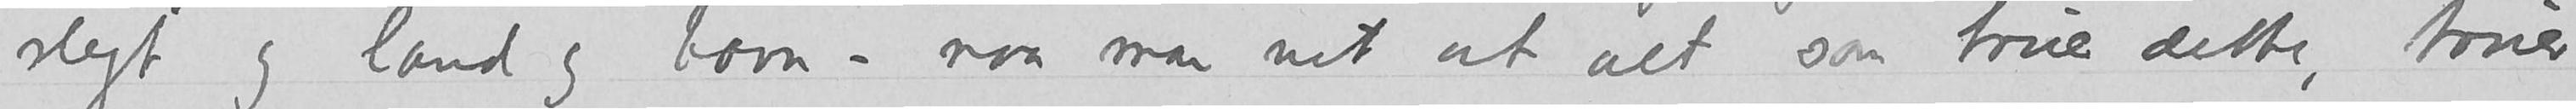slegt og land og barn – naar man vet at alt som truer dette, truer
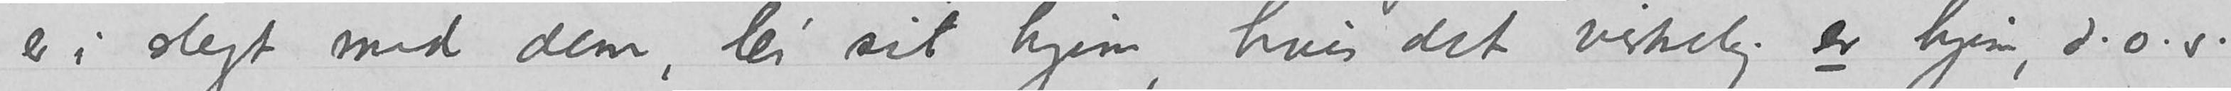er i slegt med dem, lei sit hjem, hvis det virkelig er hjem, d.v.s.
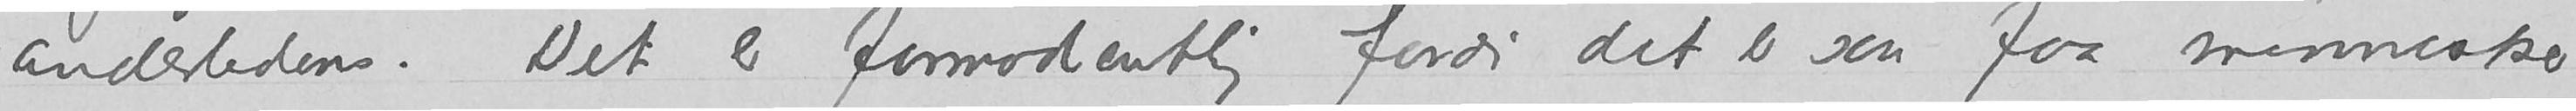anderledens. Det er formodentlig fordi det er saa faa mennesker
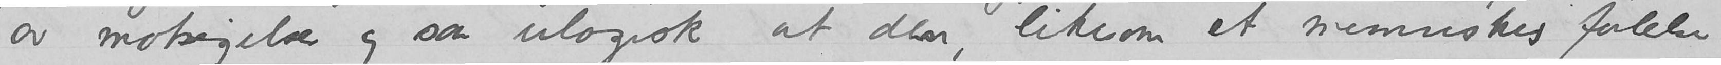av motsigelser og saa ulogisk at den, likesom et menneskes følelser
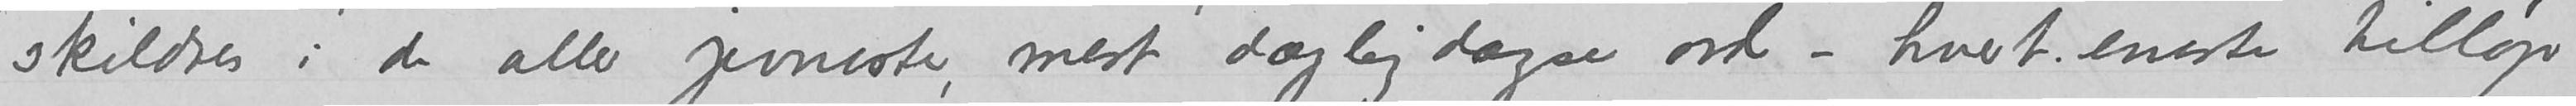skildres i de aller jevneste, mest dagligdagse ord – hvert eneste tilløp
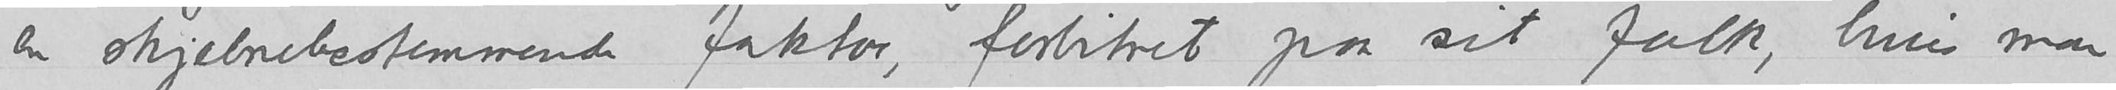en skjebnebestemmende faktor, forbitret paa sit folk, hvis man
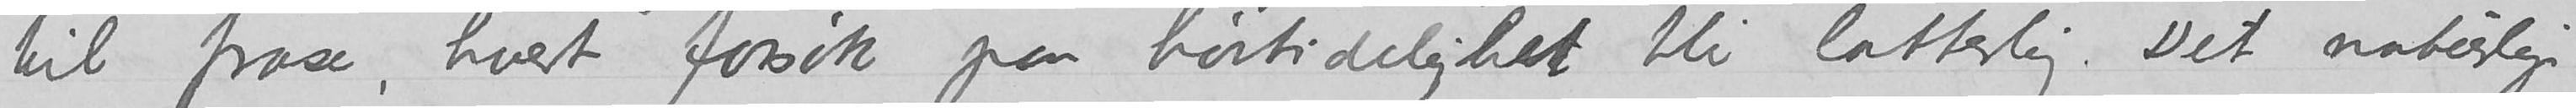til frase, hvert forsøk paa høitidelighet blir latterlig. Det naturlige
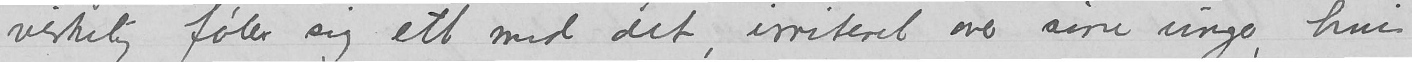virkelig føler sig ett med det, irriteret over sine unger, hvis
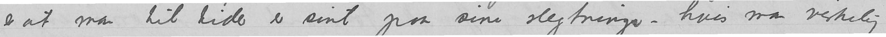er at man til tider er sint paa sine slegtninger – hvis man virkelig
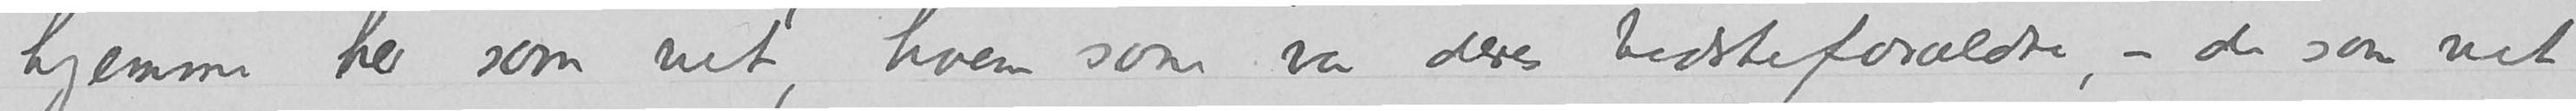hjemme her som vet, hvem som var deres bedsteforældre, - de som vet
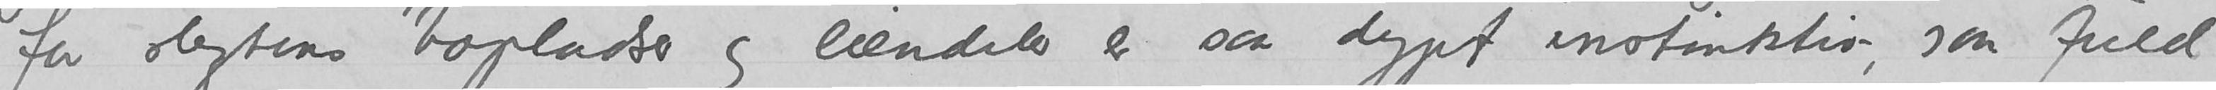for slegtens bopladser og eiendeler er saa dypt instinktiv, saa fuld
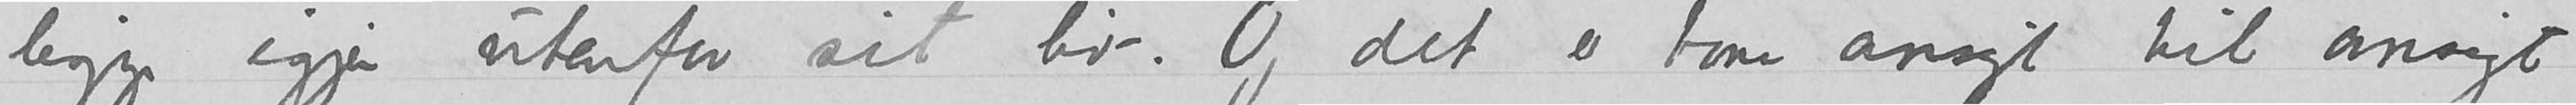ligge igjen utenfor sit liv. Og det er bare ansigt til ansigt
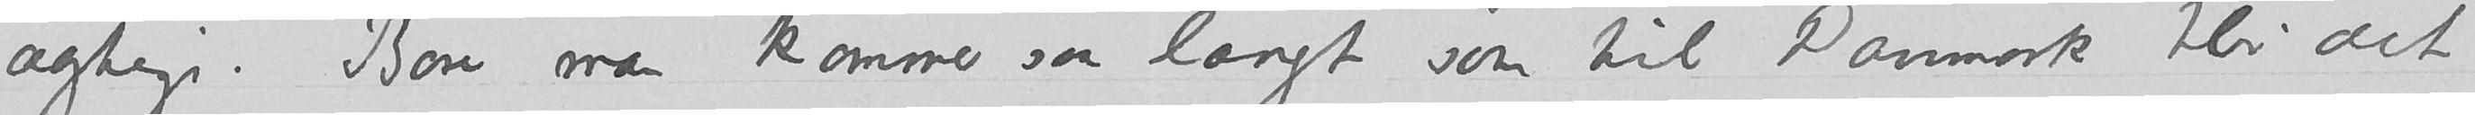agtige. Bare man kommer saa langt som til Danmark blir det
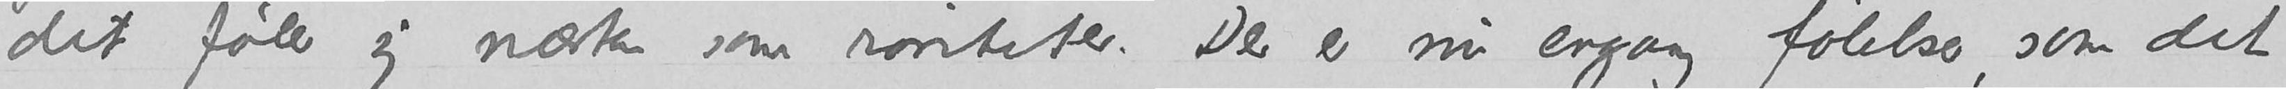det føler sig næsten som rariteter. Der er nu engang følelser, som det
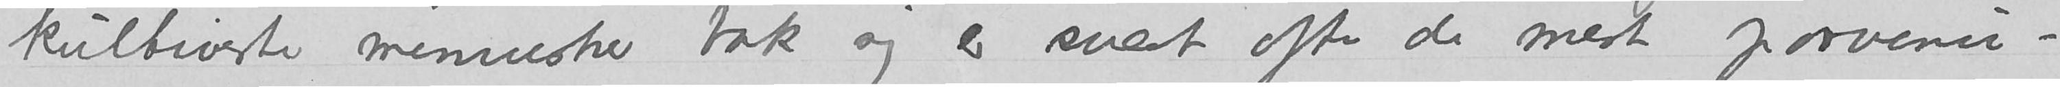kultiverte mennesker bak sig er svært ofte de mest parvenu-
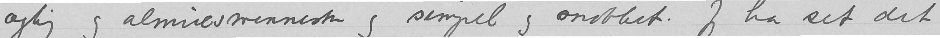agtig og almuesmenneske og simpel og snobbet. Jeg har set det
